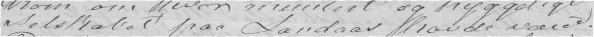selskabet paa Landaas havde været.
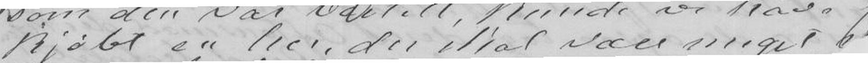kjøbt en her, der skal være meget
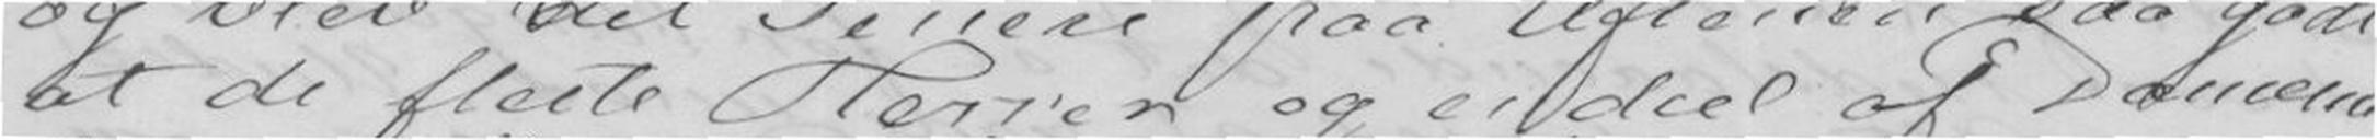at de fleste Herrer og endeel af Damerne
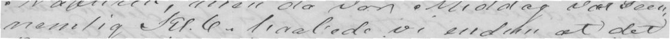nemlig Kl.6. haabede vi endnu at det
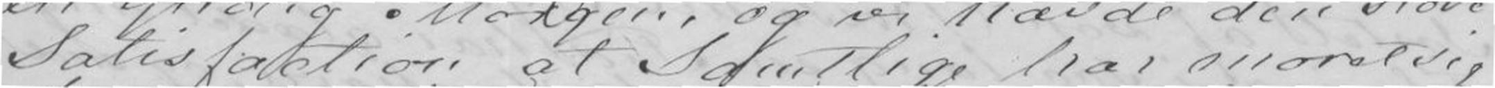Satisfaction at Samtlige har moret sig
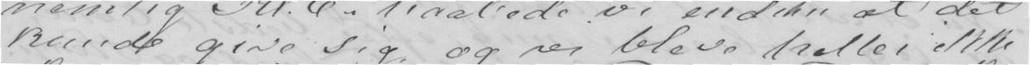kunde give sig, og vi blev heller ikke
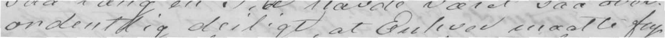ordentlig deiligt, at Enhver maatte fry-
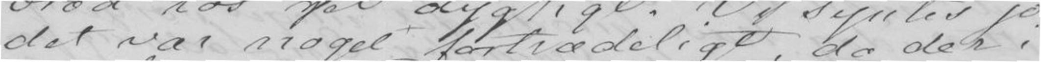det var noget fortrædeligt, da der i
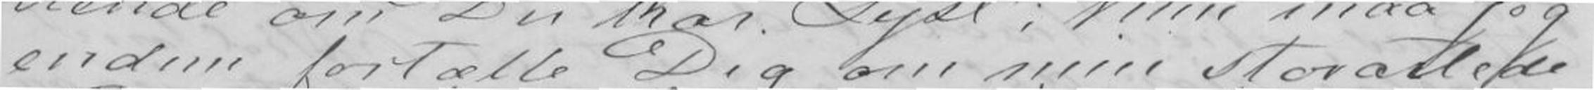endnu fortælle Dig om min Stoartede
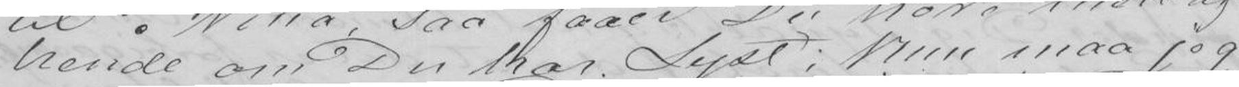hende om Du har Lyst; kun maa jeg
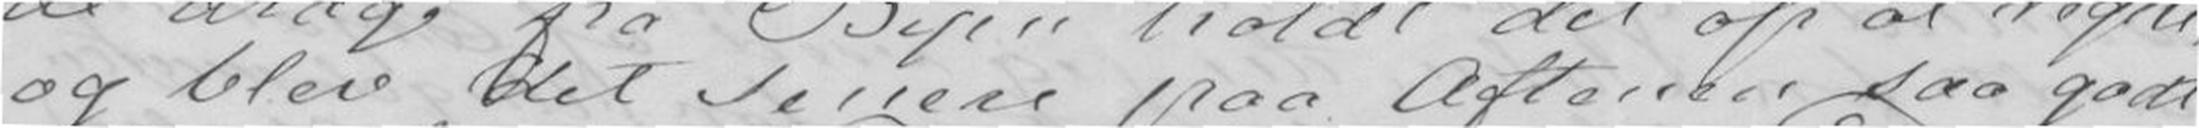og blev det senere paa Aftenen saa godt
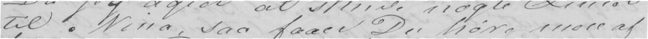til Nina, saa faaer Du høre mere af
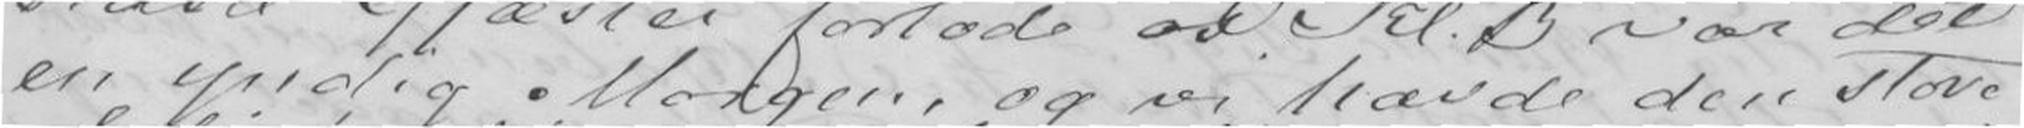en yndig Morgen, og vi havde den store
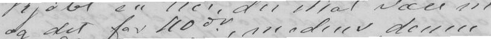og det for 110 do, medens denne
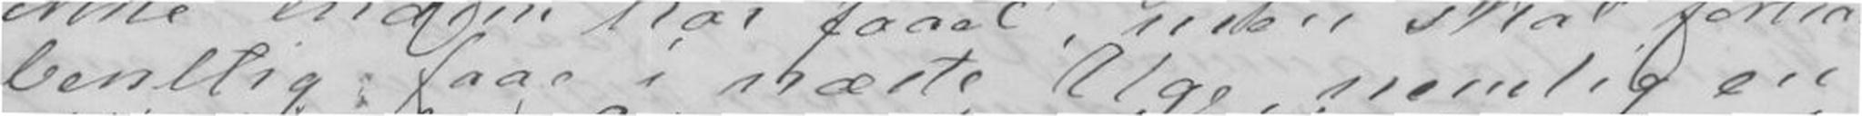bentlig faae i næste Uge, enmlig en
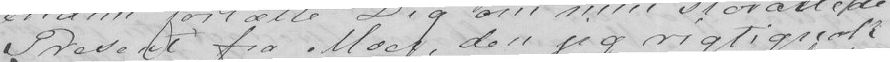Present fra Moer, den jeg rigtignok
